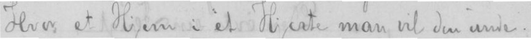Hvor et Hjem i et Hjerte man vil den unde.
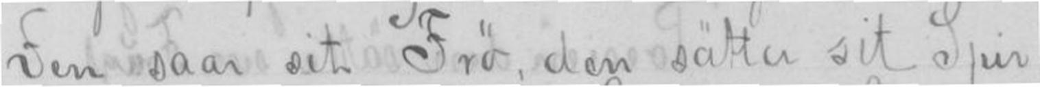Ven saar sit Frø, den sätter sit Sjin
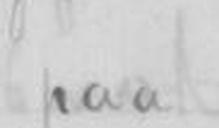paa
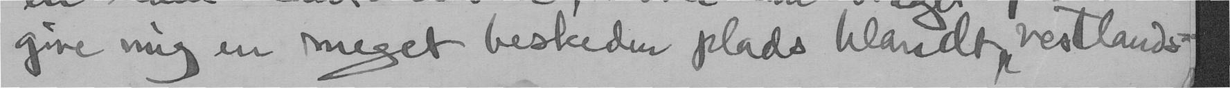give mig en meget beskeden plads blandt "vestlands-
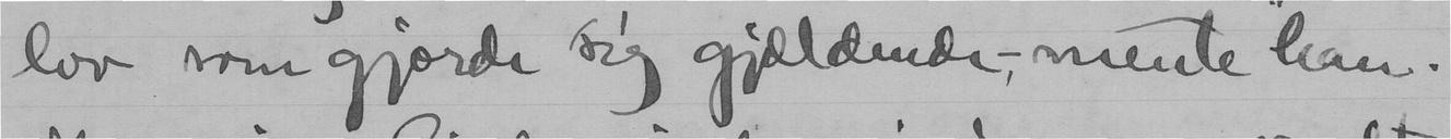lov som gjorde sig gjældende - mente han.
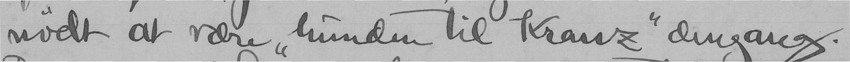nødt at bære "hunden til Kranz" dengang.
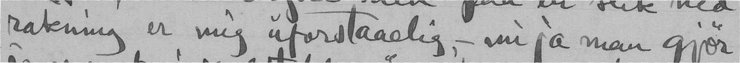rakning er mig uforstaaelig, - nu ja man gjør
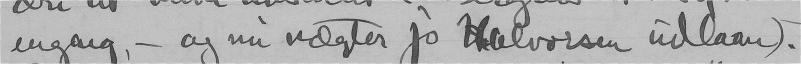engang, - og nu nægter jo Halvorsen udlaan).
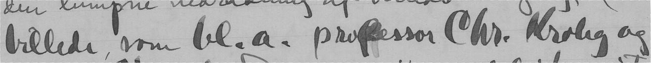billede, som ble a. professor Chr. Krohg og
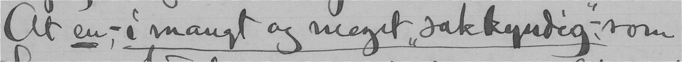At en, - i mangt og meget "sakkyndig", - som
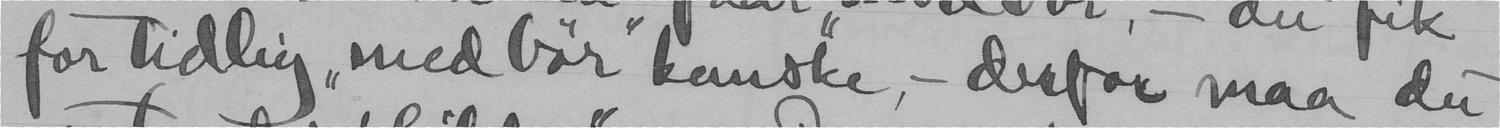for tidlig "medbør" kanske, - derfor maa du
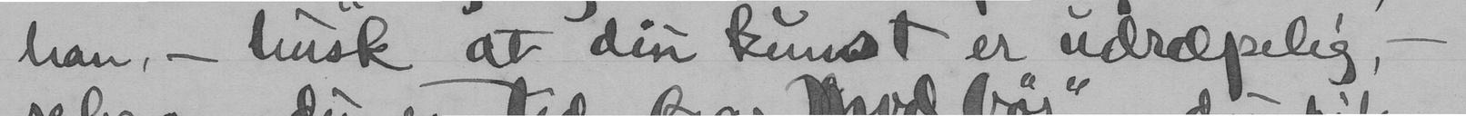han, - husk at din kunst er udræpelig, -
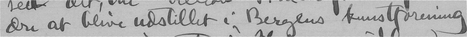ære at blive udstillet i Bergens kunstforening
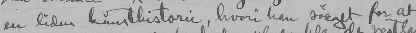en liden kunsthistorie, hvori han sørget for at
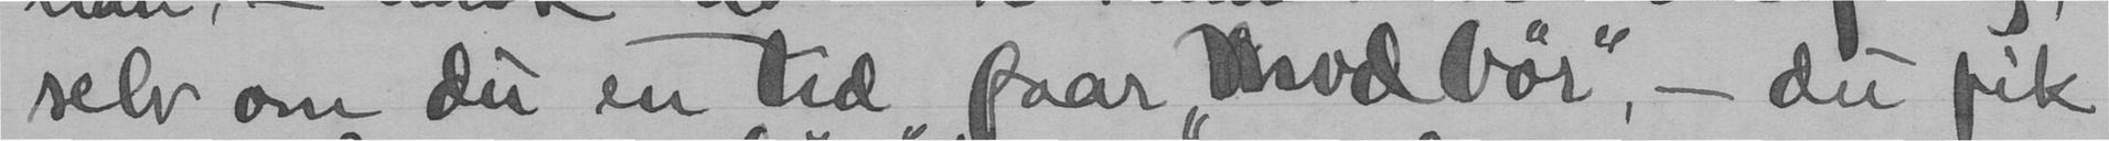selv om du en tid faar "hodbør", - der fik
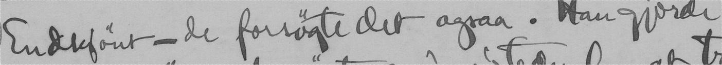Endkjønt - de forsøgte det ogsaa. Han gjorde
